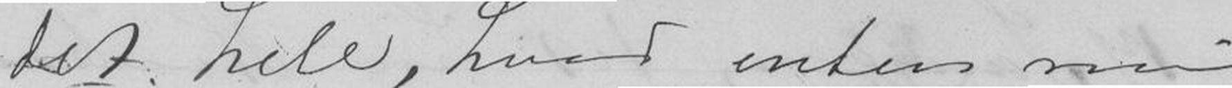det hele, hvad enten nu
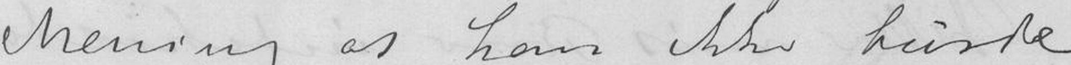Mening at ham ikke burde
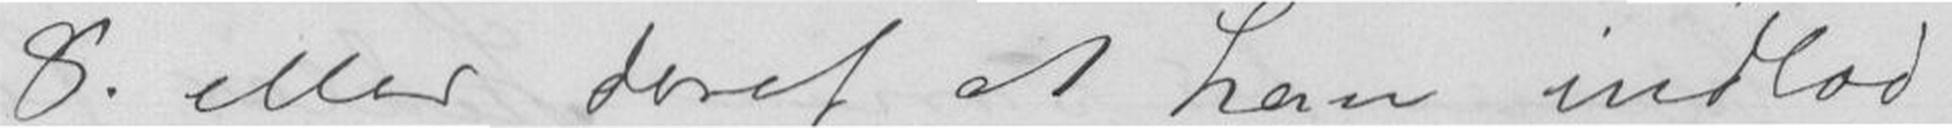8. eller deraf at han indlod
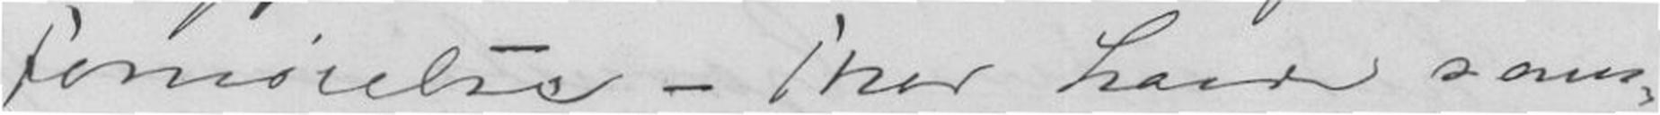Fornøielse - Thor havde sam-
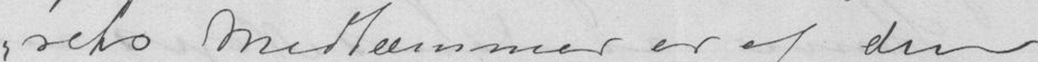rets Medlæmmer er af den
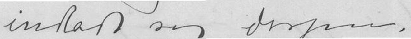indladt sig derpaa.
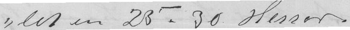let en 25 - 30 Herrer.
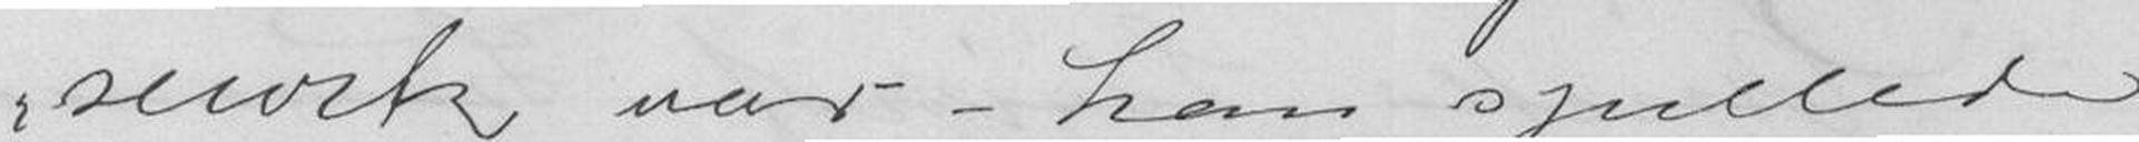sewik var - han spillede
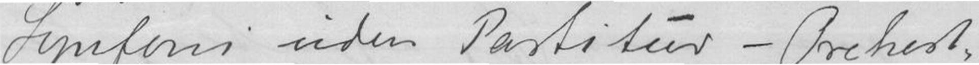Synfoni uden Patitur - Orchest-
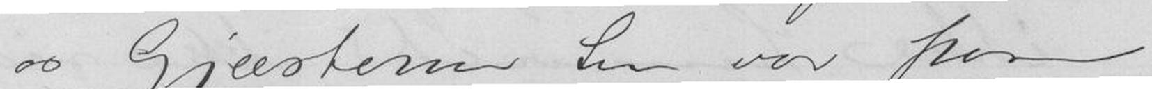os Gjesterne til vor store
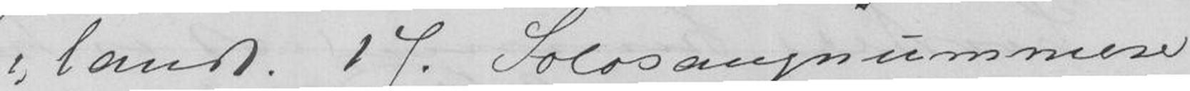landt 17 Solosangnummere
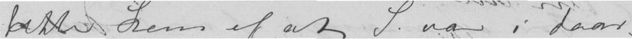dette kom af at S. var i daar-
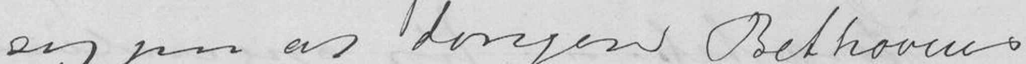sig paa at dirigere Bethovens
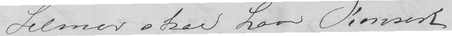Selmer skal have Konsert
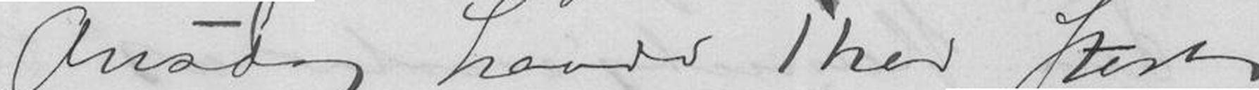Onsdag havde Thor stort
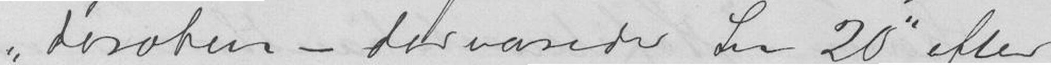deroben - der varede til 20" efter
